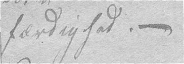færdighed.–
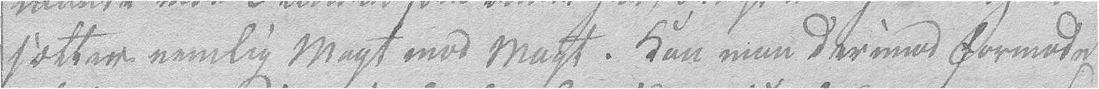sætter nemlig Magt mod Magt. Kan man derimod formode
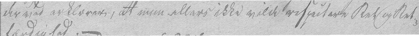derved erklærer, at man ellers ikke vilde respectere Ret og Ret-
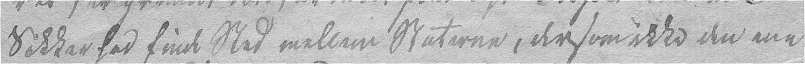Sikkerhed finde Sted mellem Staterne, dersom ikke den ene
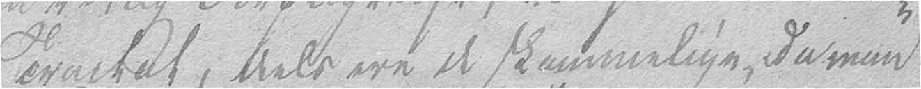Tractat, deels ere de skammelige, da man
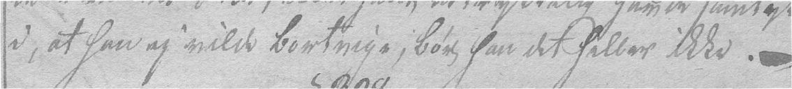i, at han ey vilde bortvige, bør han det heller ikke. –
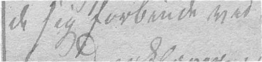de sig forbinde ved
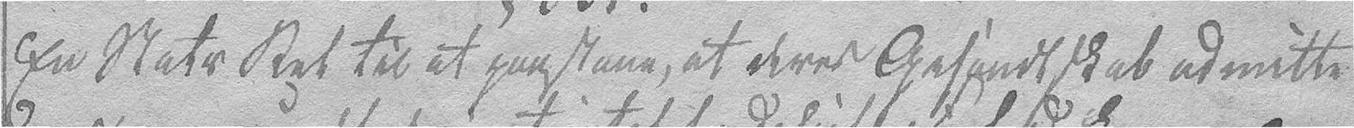En Stats Ret til at paastaae, at deres Gesandtskab admitte-
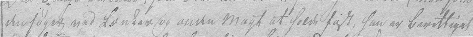den søger ved Lænker og anden Magt at holde fast, han er berettiget
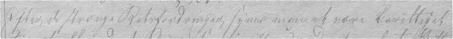Efter de strænge Retsfordringer synes man at være berettiget
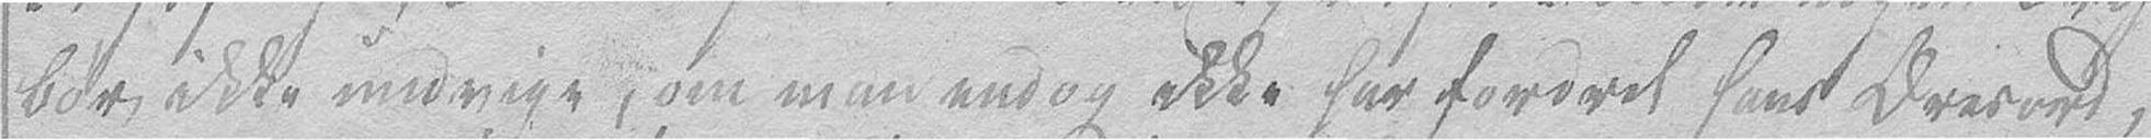bør ikke undvige, om man endog ikke har fordret hans Æresord,
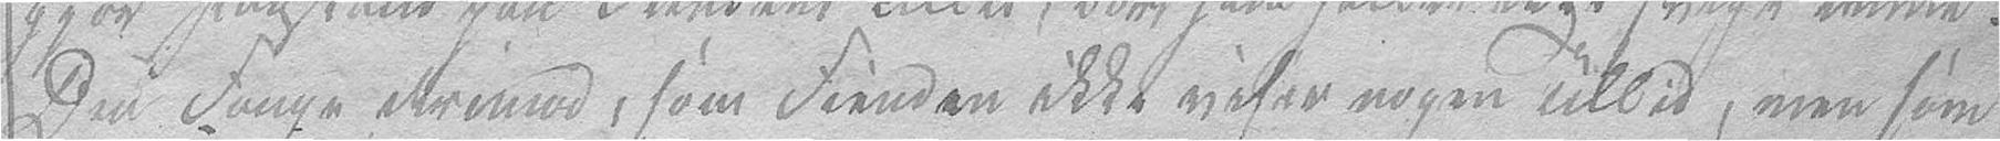Den Fange derimod, som Fienden ikke viser nogen Tillid, men som
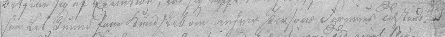saa let kunne have Kundskab om enhver Persons Formues Tilstand.
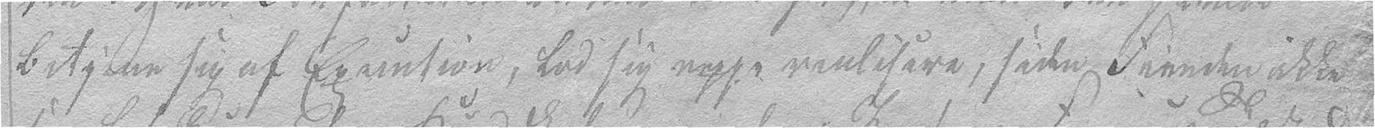betjene sig af Execution, lod sig neppe realisere, siden Fienden ikke
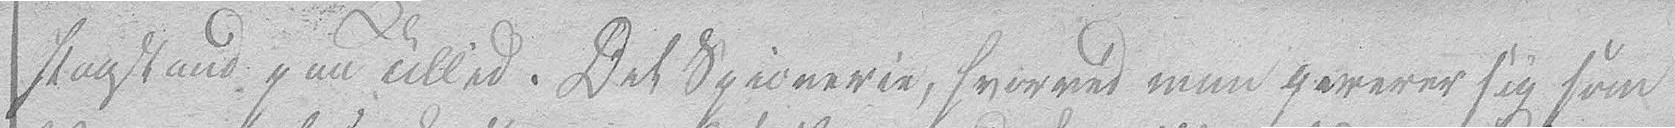Paastand paa Tillid. Det Spionerie, hvorved man gererer sig som
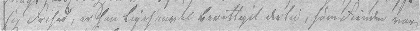sig Frihed, er han ligesaavel berettiget dertil, som Fienden var
